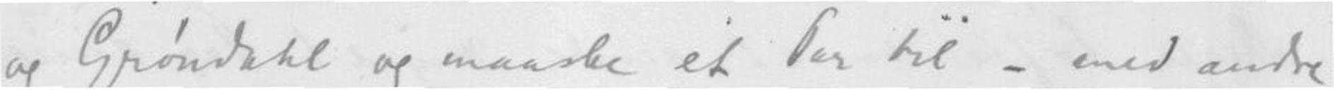og Grøndahl og maaske et Var til - med andre
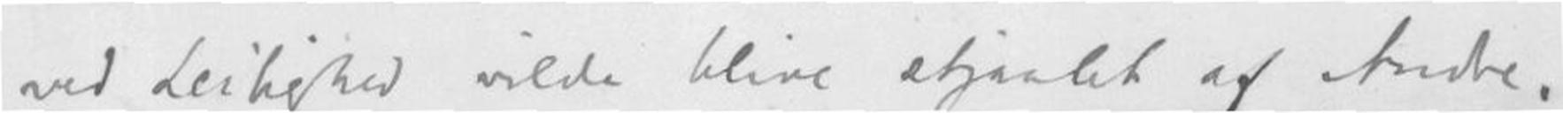ved Leilighed vilde blive stjaalet af Andre.
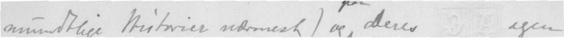mundtlige Historier nærmest) og, Deres egen
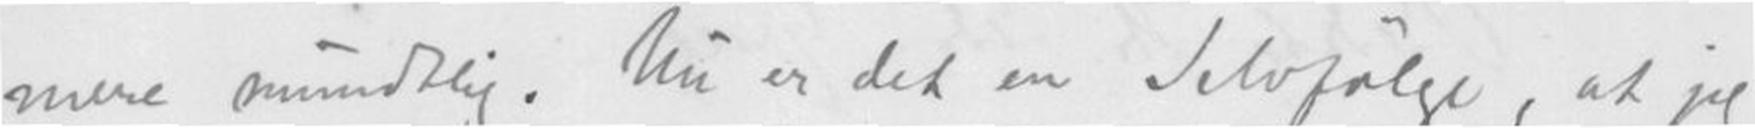mere mundtlig. Nu er det en Selvfølge, at jeg
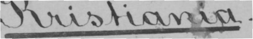Kristiania.
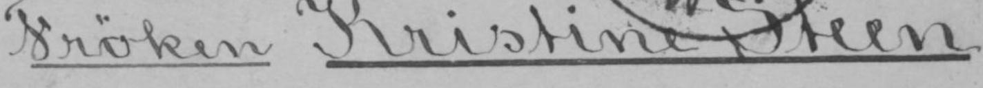Frøken Kristine Steen.
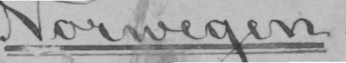Norwegen.
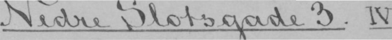Nedre Slotsgade 3. IV.
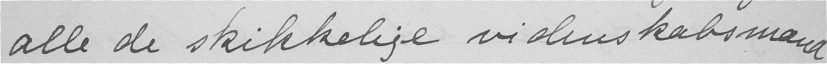alle de skikkelige videnskabsmænd
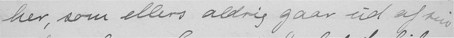her, som ellers aldrig gaar ud af sin
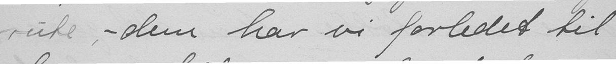rute - dem har vi forledet til
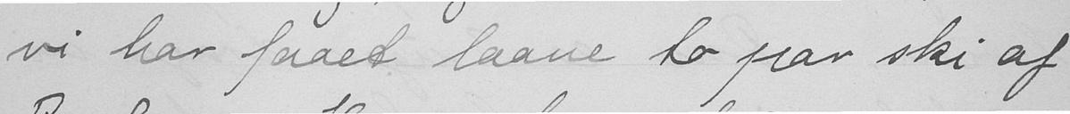vi har faaet laane to par ski af
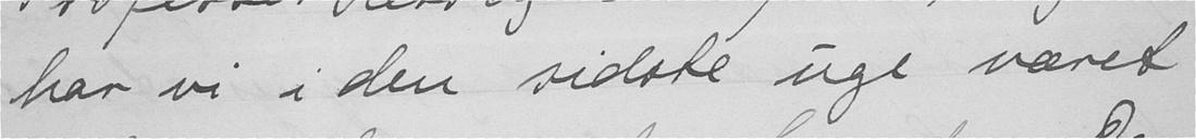har vi i den sidste uge været
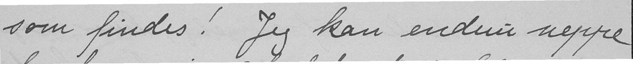som findes! Jeg kan endnu neppe
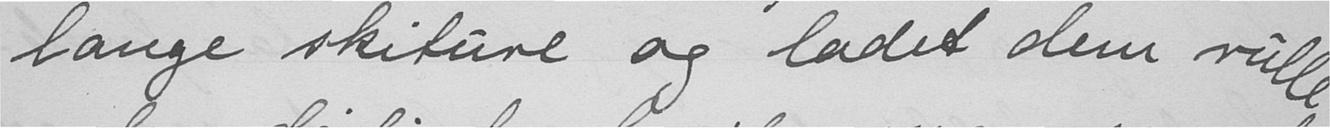lange skiture og ladet dem rulle
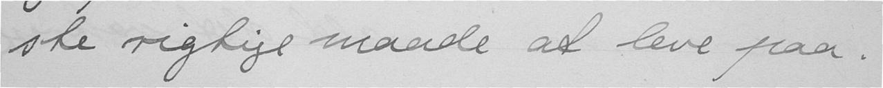ste rigtige maade at leve paa.
